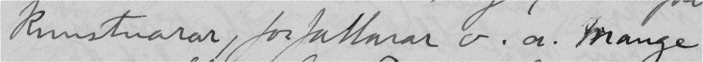kunstnarar, forfattarar o.a. Mange
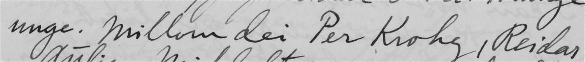unge. Millom dei Per Krohg, Reidar
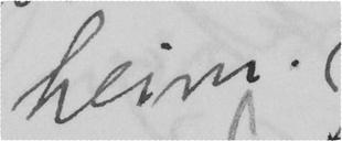heim.
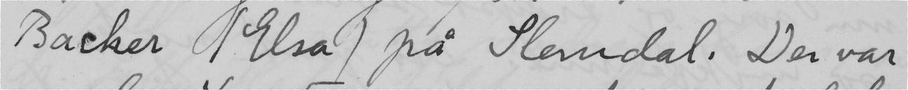Backer (Elsa) på Slemdal. Der var
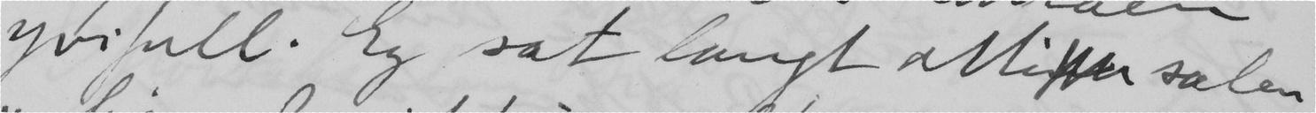yvifull. Eg sat langt atti salen
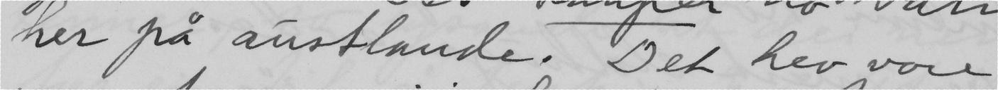her på austlande. Det hev vore
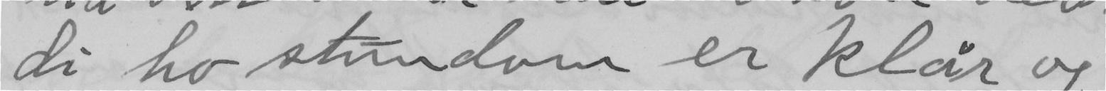di ho stundom er klår og
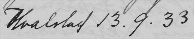Hvalstad 13.9.33
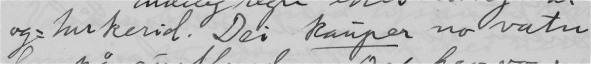og turkerid. Dei kauper no vatn
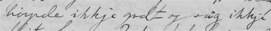höyrde ikkje godt og såg ikkje
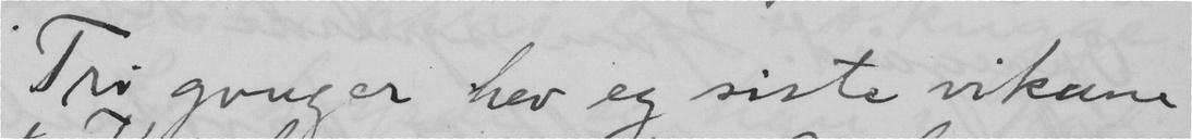Tri gonger hev eg siste vikane
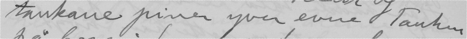tankane piner yver evne tanken
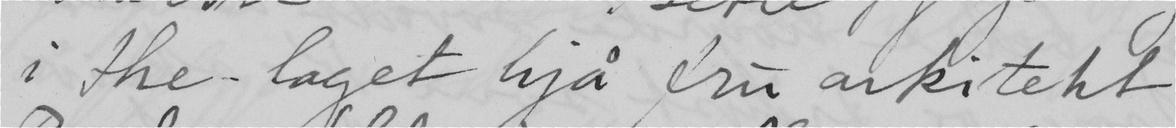i the-laget hjå fru arkitekt
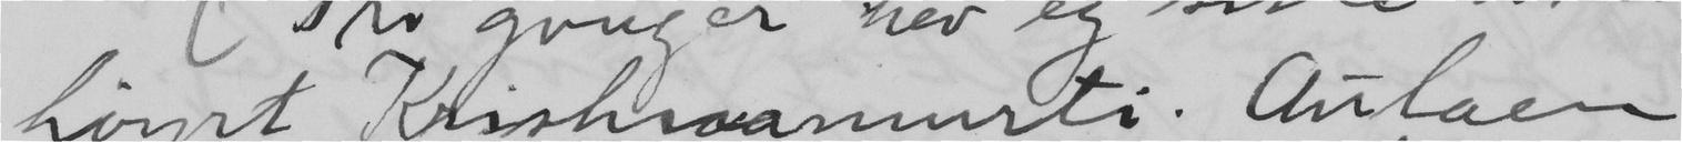höyrt Krishnamurti. Aulaen
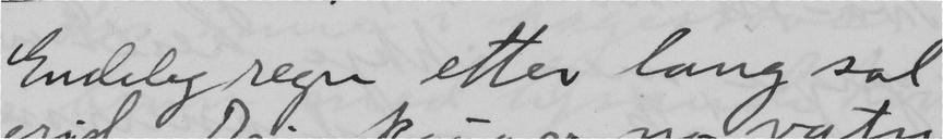Endeleg regn etter lang sol
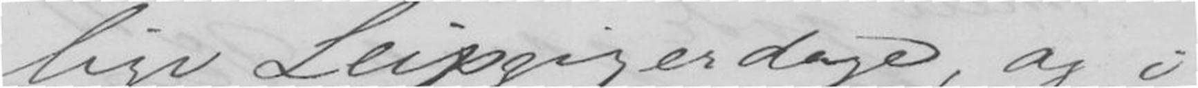lige Leipzigerdage, og i
